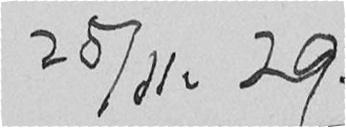25/11. 29.
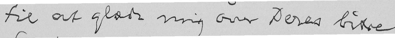til at glæde mig over Deres bitre
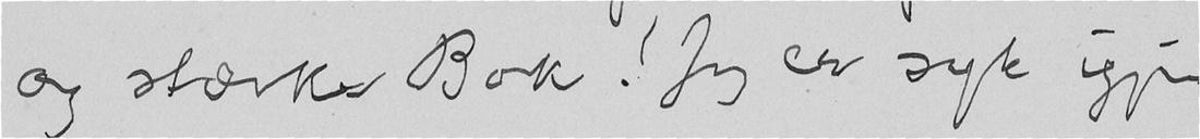og stærke Bok! Jeg er syk igjen
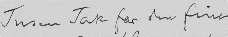Tusen Tak for den fine
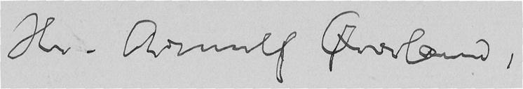Hr. Arnulf Øverland,
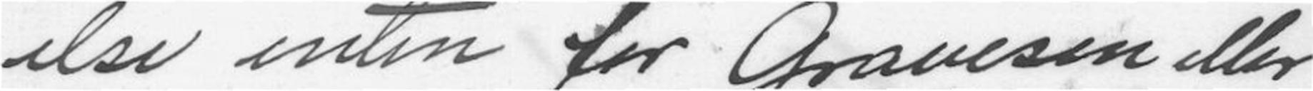else enten for Gravesen eller
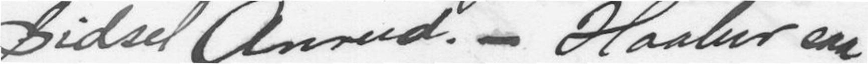Sidsel Ånrud. - Haaber saa
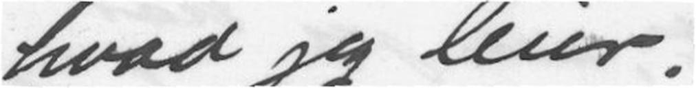hvad jeg leier.
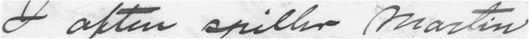I aften spiller Martin
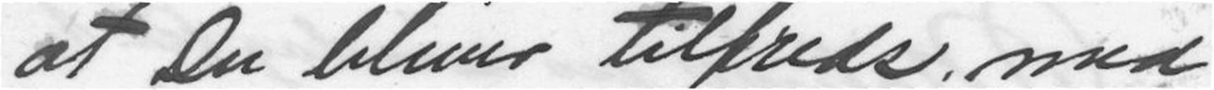at Du bliver tilfreds, med
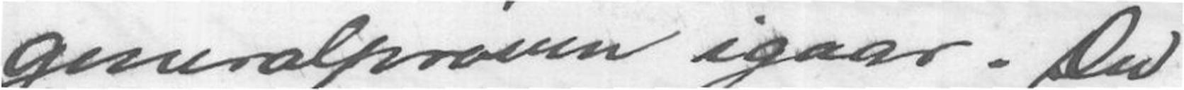generalprøven igaar. Du
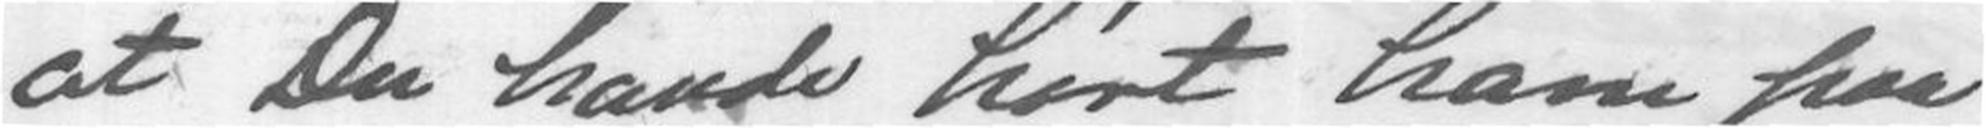at Du havde hørt ham paa
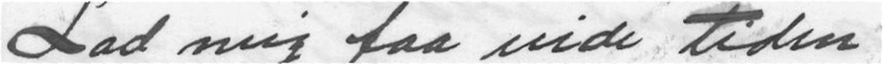Lad mig faa vide tiden
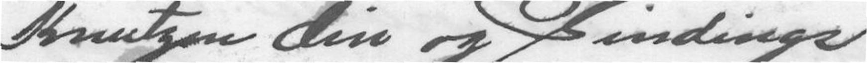Knudzen din og Sindings
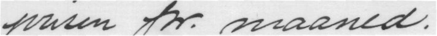prisen pr. maaned.
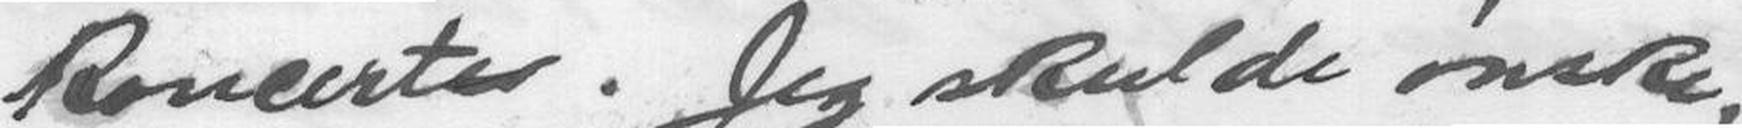konserter. Jeg skulde ønske,
